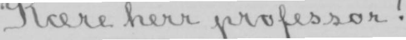Kære herr professor!
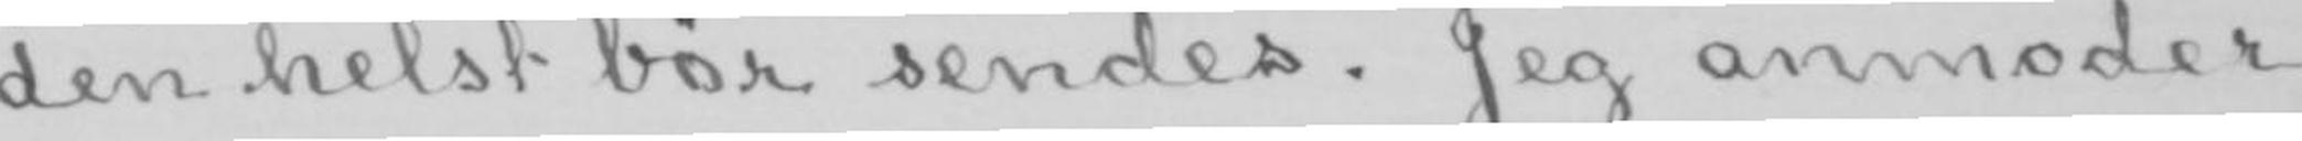den helst bør sendes. Jeg anmoder
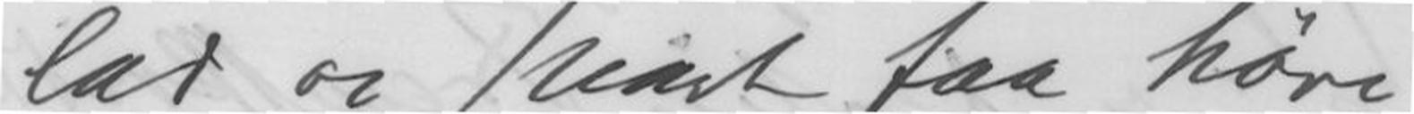lat os snart faa høre
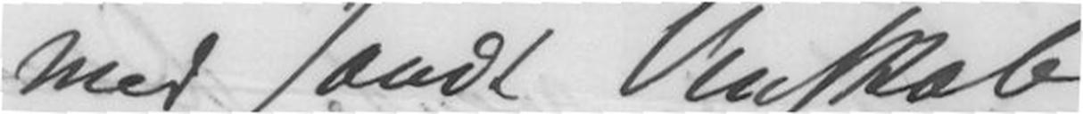med sandt Venskab
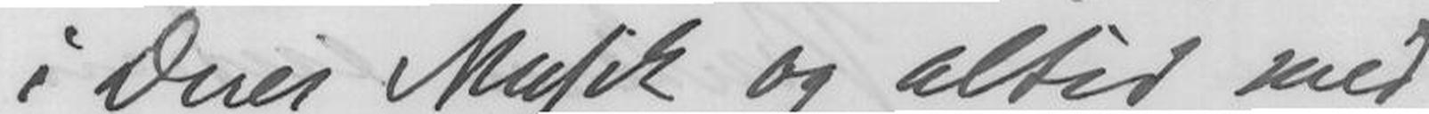i Deres Musik og altid med
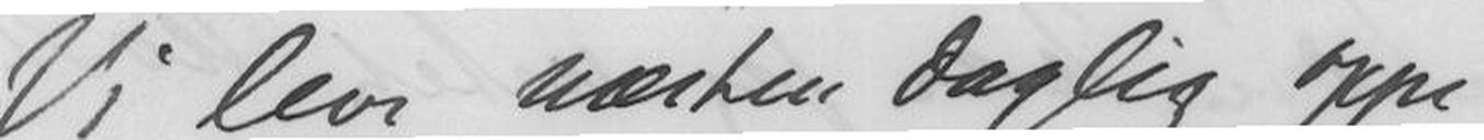Vi lever næsten daglig oppe
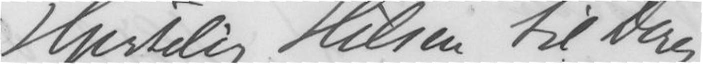Hjertelig Hilsen til Deres
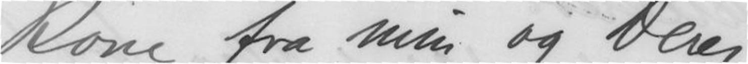Kone fra min og Deres
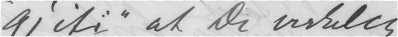"gjiti" at De virkelig
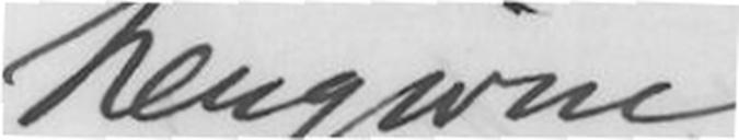hengivne
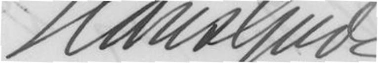Hans Gude
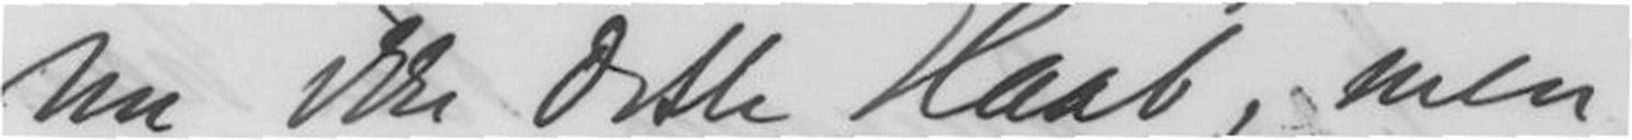nu ikke dette Haab, men
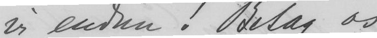vi endnu! Belag os
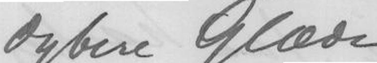dybere Glæde
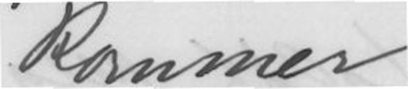kommer
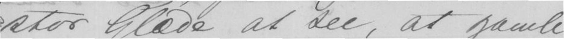stor Glæde at see, at gamle
